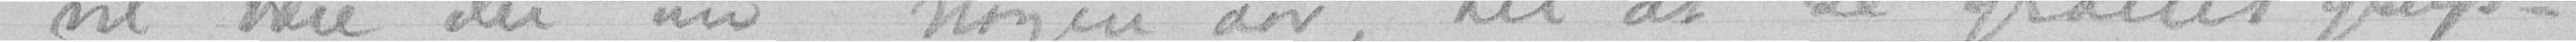vil være der nu nogen aar, til at de granitgrup-
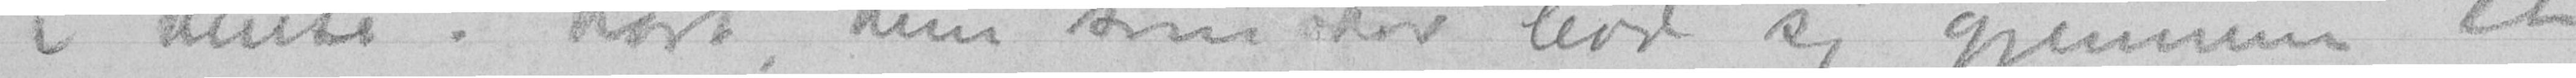i vente. Rart hun som har levd sig gjennem et
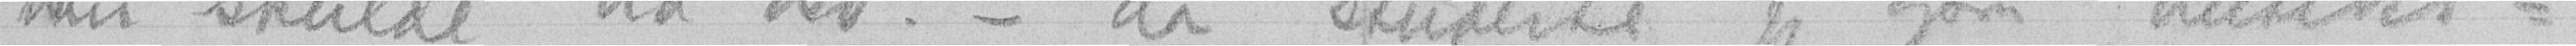han skulde ha osv. - da studerte jeg ogsaa butiks-
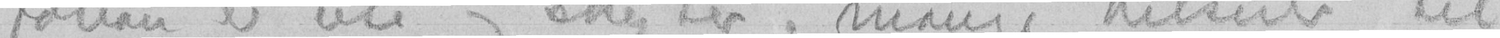Johan er ute og skyter; mange hilsner til
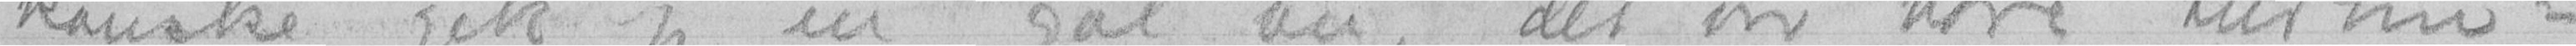kankse gik jeg en gal vei, det er bare turbin-
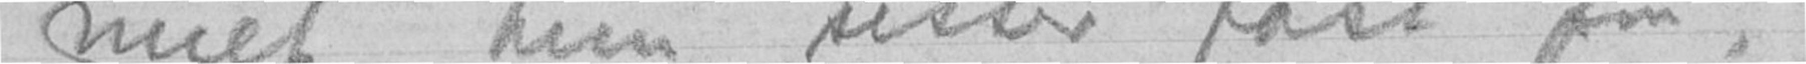nuet hun sitter fast paa,
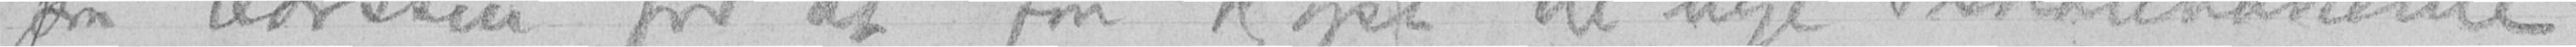paa Carsten for at faa kjøpt de nye skolebøkene
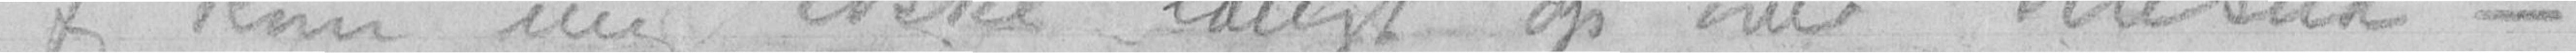Jeg kom mig ikke langt op over Nesna -
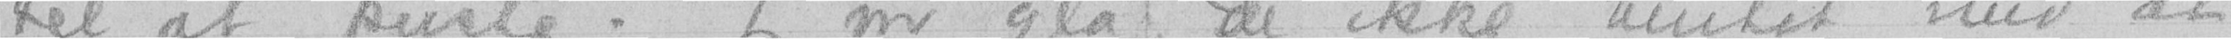til at puste. Jeg var gla, De ikke ventet med at
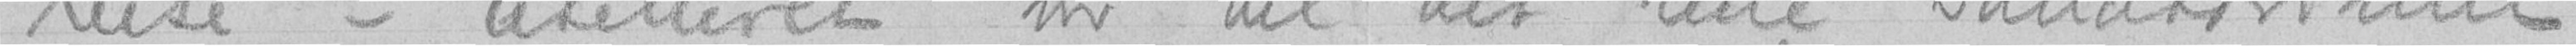reise - atelieret er vel det rene sanatorium
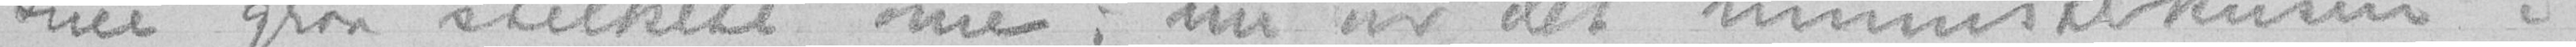sine graa stilkete øine; nu er det ministerkrisen i
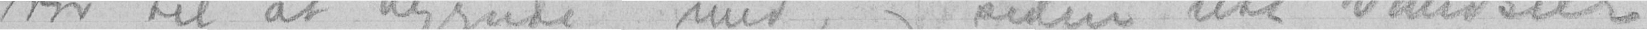rør til at begynde med, og siden litt vandsiler
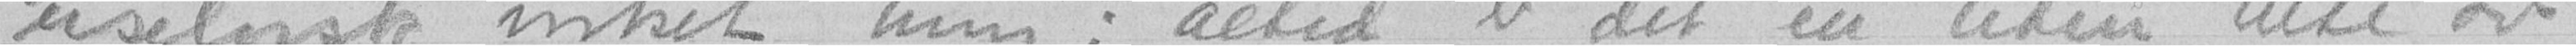uselvisk virket hun; altid er det en liten bete av
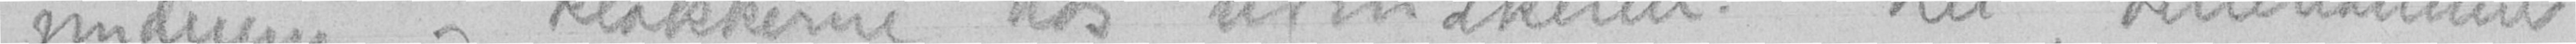vinduene og klokkerne hos urmakeren. Nei, Lillehammer
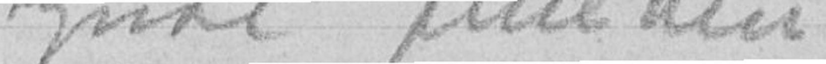tynde flueben
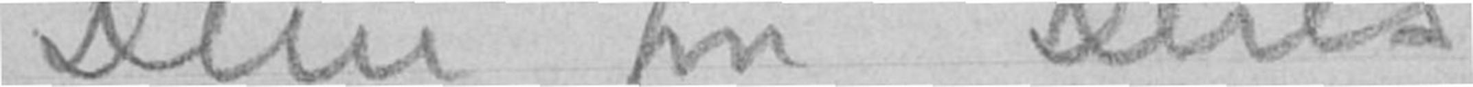Dem fra Deres
